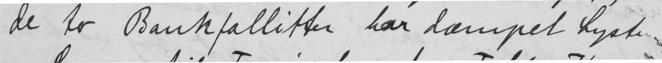de to Bankfallitter har dæmpet Lyst
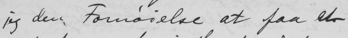jeg den Fornøielse at faa et
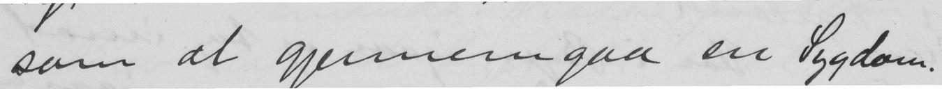som at gjennemgaa en sygdom.
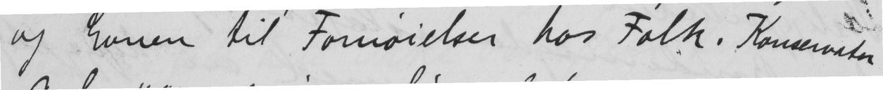og Evnen til Fornøielser hos Folk. Konservator
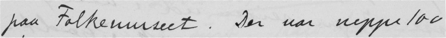paa Folkemuseet. Der var neppe 100
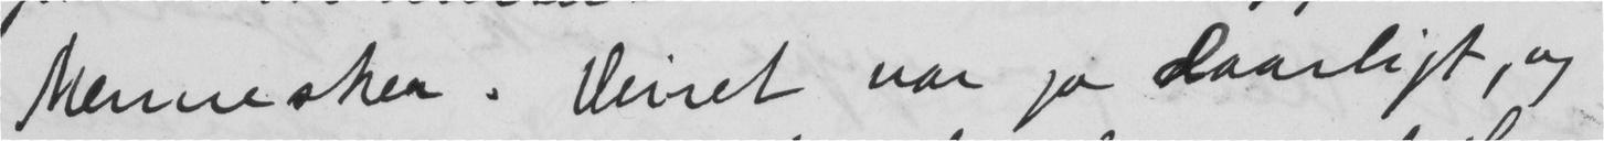Mennesker ; Veiret var jo daarligt, og
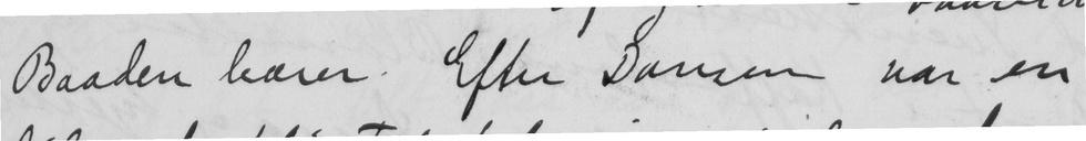Baaden bærer. Efter Dansen var en
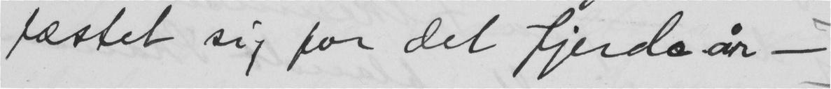fæstet sig for det fjerde år -
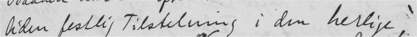liden festlig Tilstelning i den herlige,
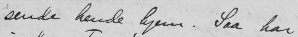sende hende hjem. Saa har
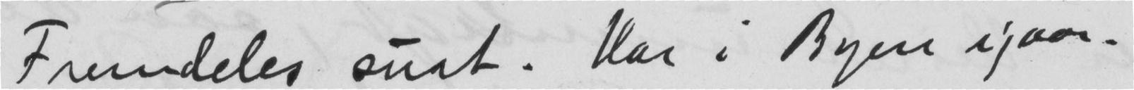Frundeles surt. Var i Byen igaar.
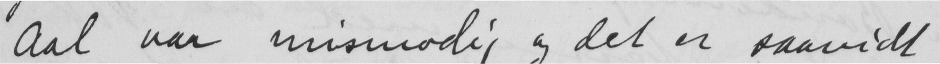Aal var mismodig og det er saavidt
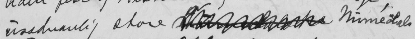usædvanlig store  Numedals
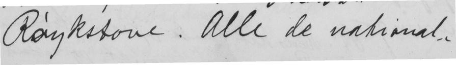Røykstove. Alle de national-
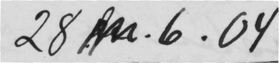28 .6.04
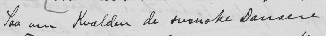Saa om Kvælden de svenske Dansere
